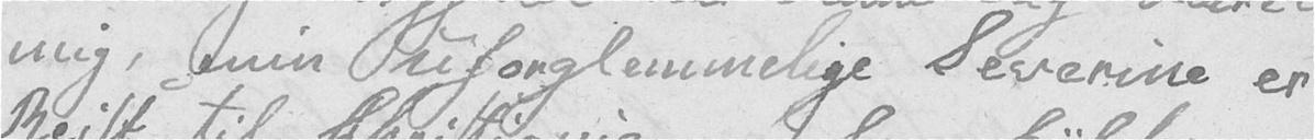mig, min uforglemmelige Severine er
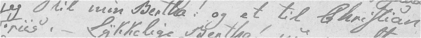jeg til min Bertha! og et til Christian
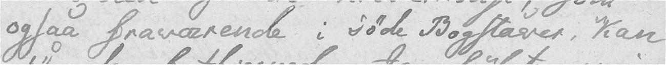ogsaa fraværende i søde Bogstaver, kan
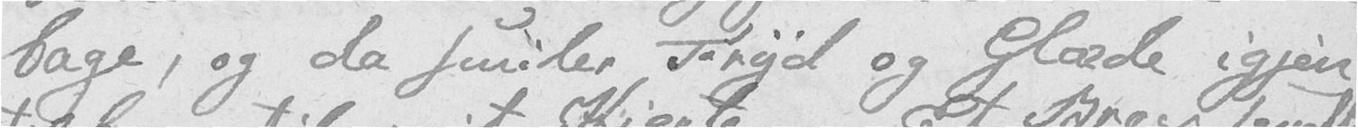bage, og da smiler Fryd og Glæde igjen
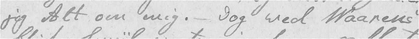jeg Alt om mig. – Dog ved Vaarens
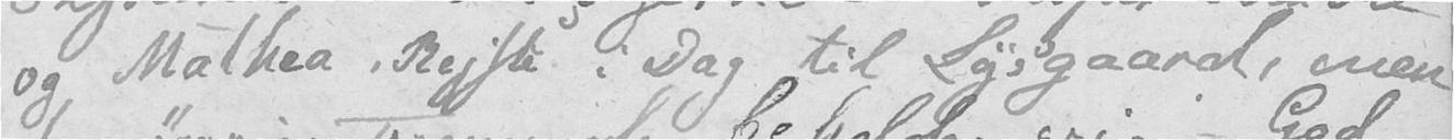og Mathea Rejste i Dag til Lysgaard, men
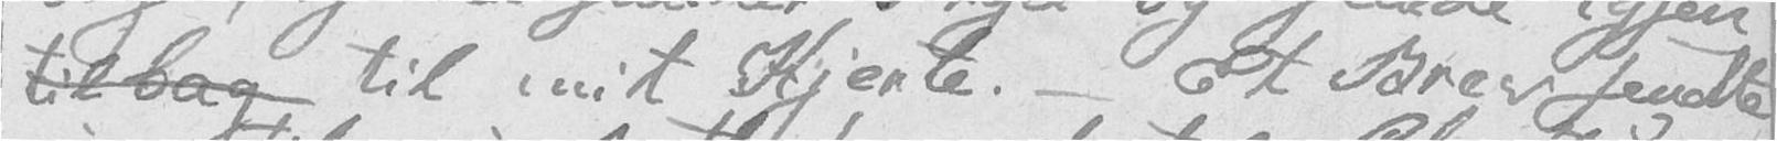tilbag til mit Hjerte. – Et Brev sendte
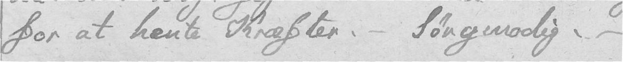for at hente Kræfter. – Sørgmodig. –
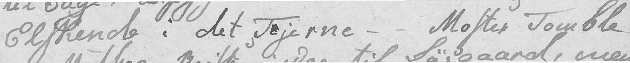Elskende i det Fjerne. – Moster Tomble
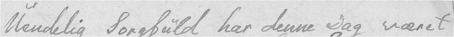Uendelig Sorgfuld har denne Dag været
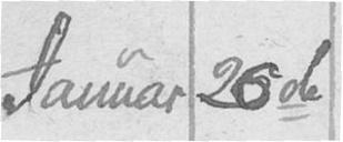Januar 26de
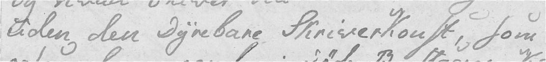uden den Dyrebare Skriverkonst, som
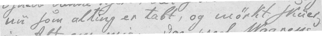nu som alting er tabt, og mørkt skuer
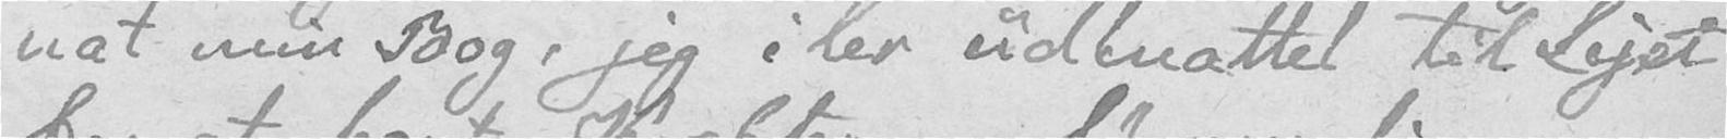nat min Bog, jeg iler udmattet til Lejet
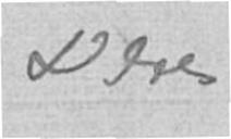Deres
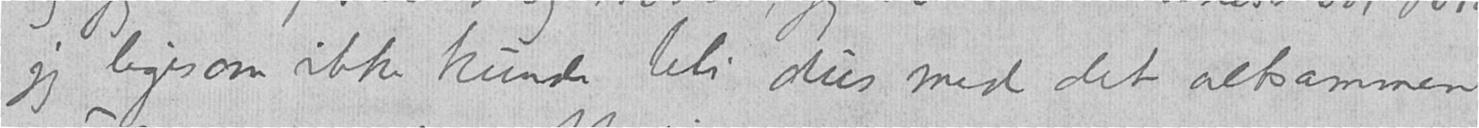jeg ligesom ikke kunde bli dus med det altsammen
